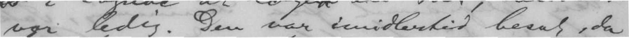var ledig. Den var imidlertid besat, da
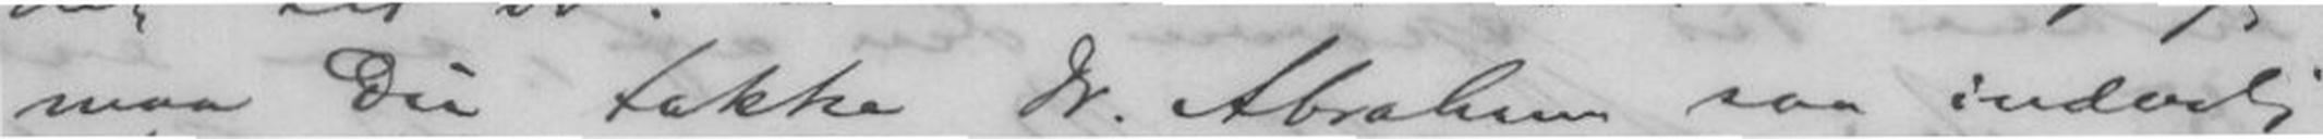maa Du takke Dr. Abraham saa inderlig
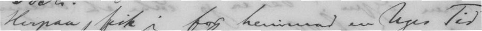Herpaa fik jeg for henimod en Uges Tid
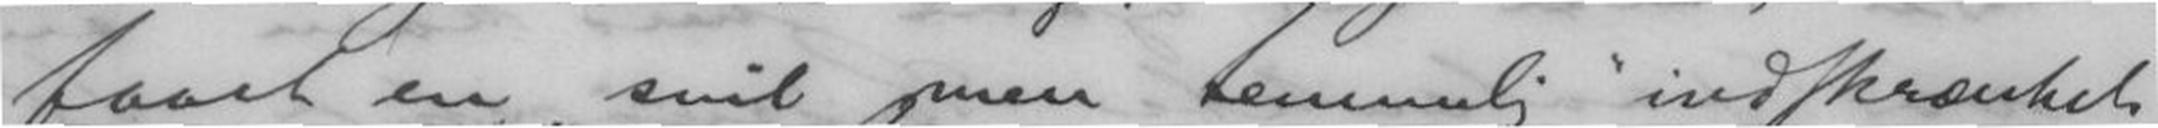faaet en snil men temmelig "indskrænket"
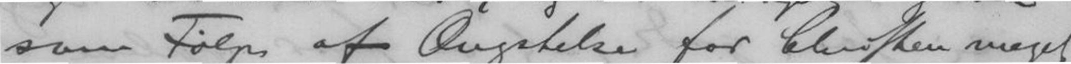som Følge af Ængstelse for Christen meget
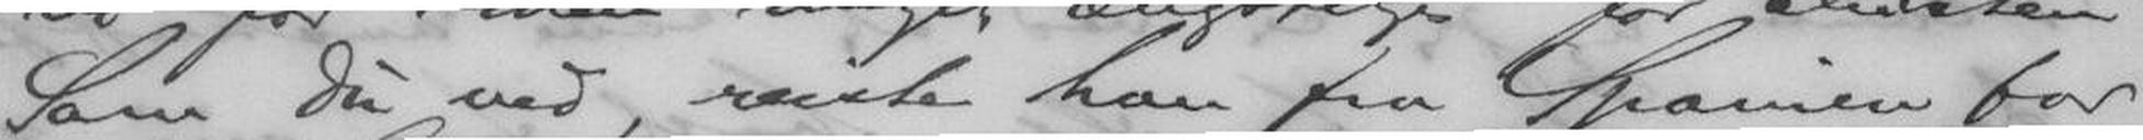Som du ved, reiste han fra Spanien for
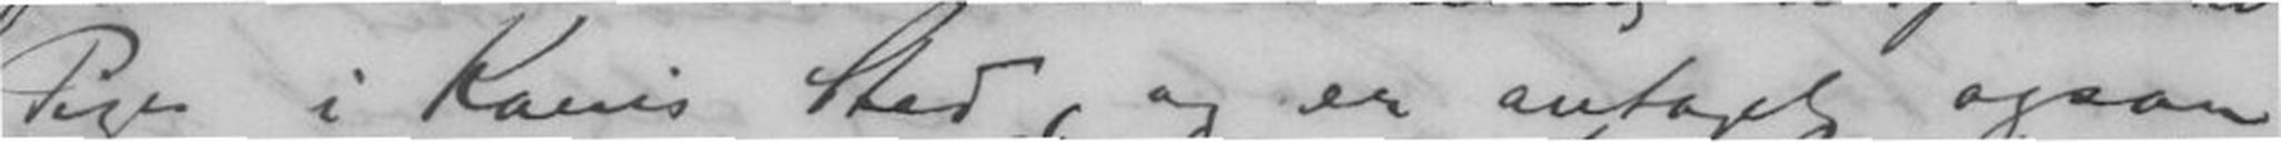Pige i Karis Sted, og er antaget ogsaa
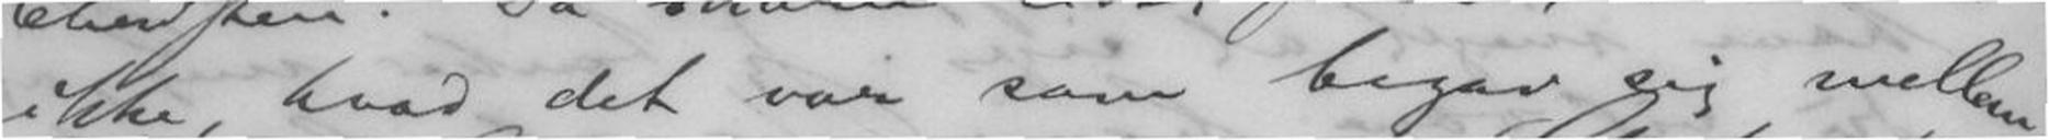ikke, hvad det var som begav sig mellem
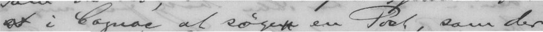at i Cognac at søge en Post, som der
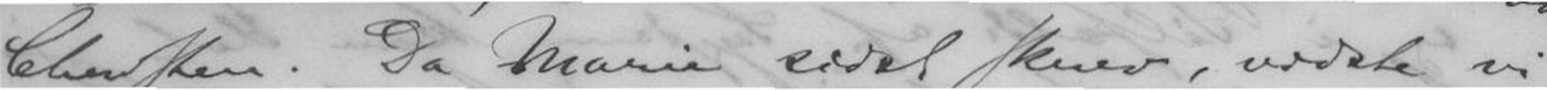Christen. Da Marie sidst skrev, vidste vi
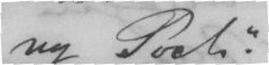ny Post".
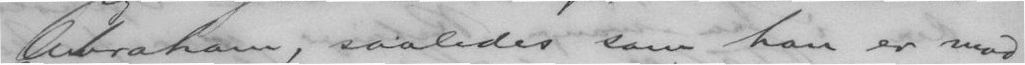Abraham, saaledes som han er mod
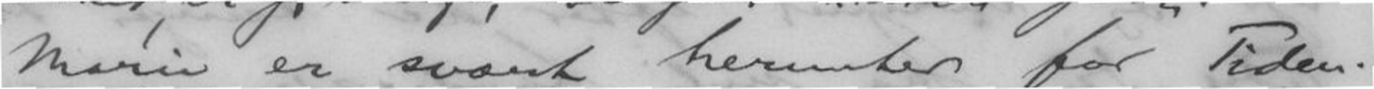Marie er svært herunter for Tiden.
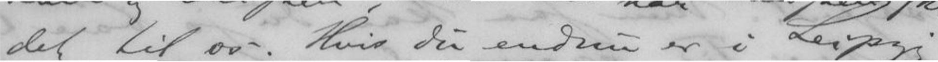det til os. Hvis du endnu er i Leipzig
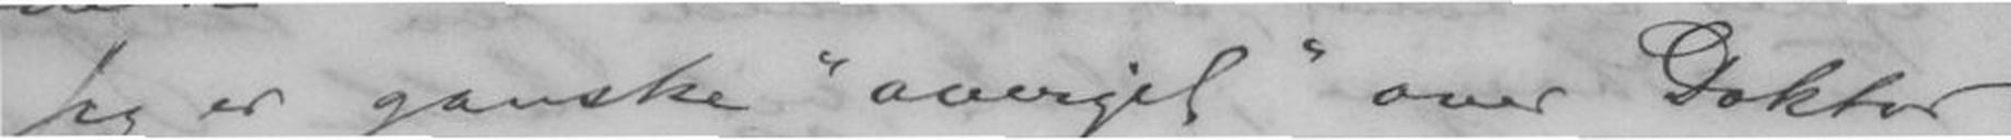Jeg er ganske "overgit" over Doktor
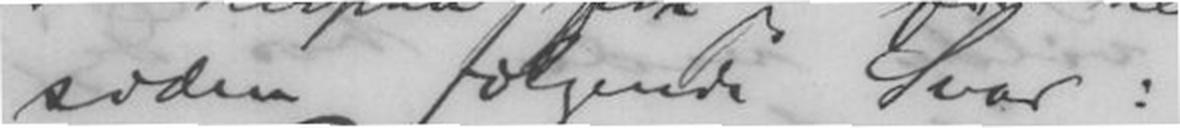siden følgende Svar:
